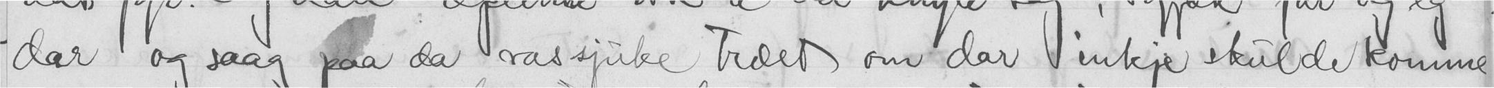dar og saag paa da rassjuke træet om dar inkje skulde komme
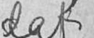dat
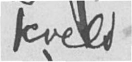kveld
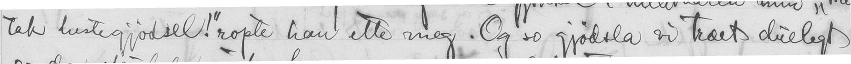tek hestegjødsel!" ropte han ette meg . Og so gjødsla vi træet duelegt
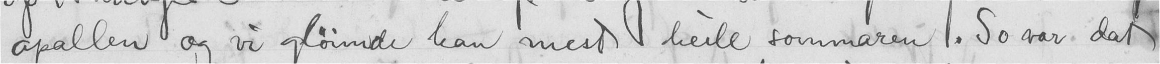apallen og vi gløimde han mest heile sommaren. So var dat
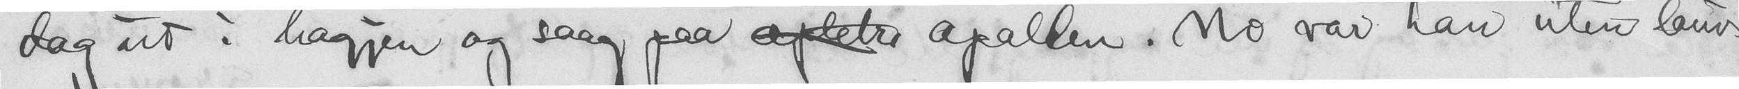dag ut i hagjen, og saag paa  apallen. No var han uten laus
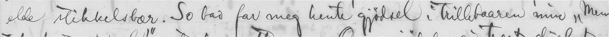elle stikkelsbær. So bad far mig hente gjødsel i trillebaaren min "Men
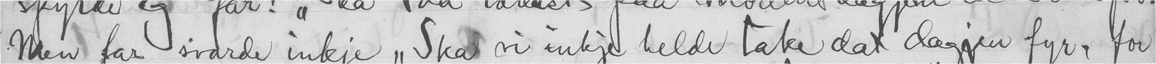Men far svarde inkje "Ska vi inkje helde take dat dagjen fyr, for
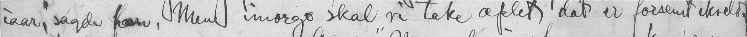iaar, sagde far. Men imorgo skal vi take æplet dat er forseint ikveld.
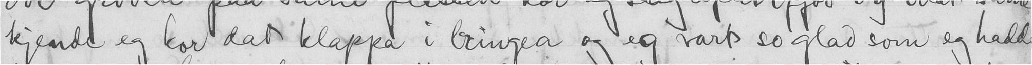kjende eg kor dat klappa i bingea og eg vart so glad som eg hadde
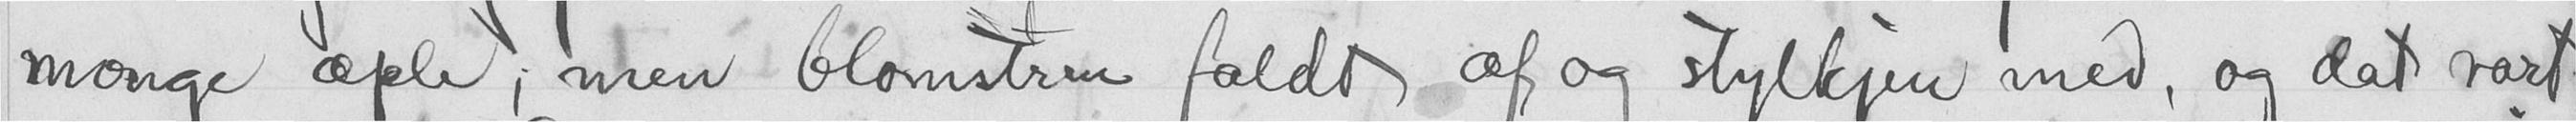monge æple; men blomstren faldt af og stylkjen med, og det vart
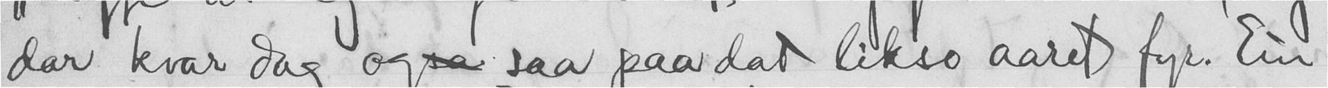dar kvar dag og saa paa dat likso aaret fyr. Ein
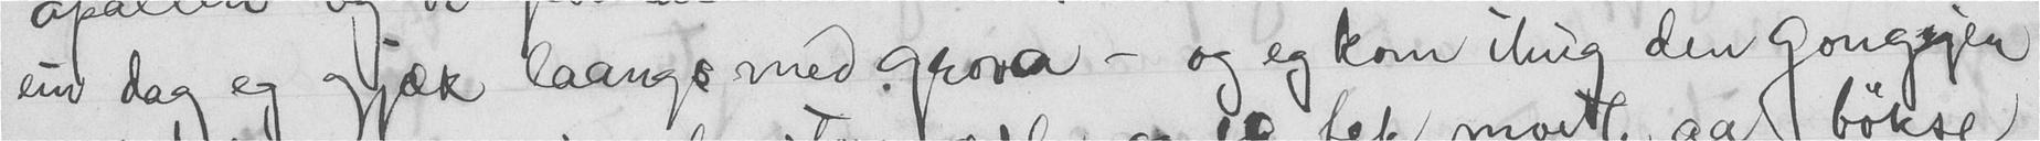ein dag eg gjæk laangs med grova - og og kom ihug den gongjen
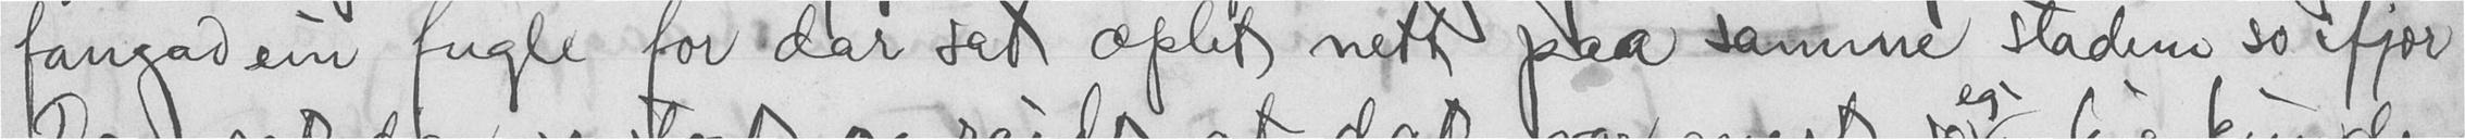fangad ein fugle for dar sat æplet nett paa samme staden so ifjor
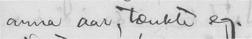anna aar, tænkte eg.
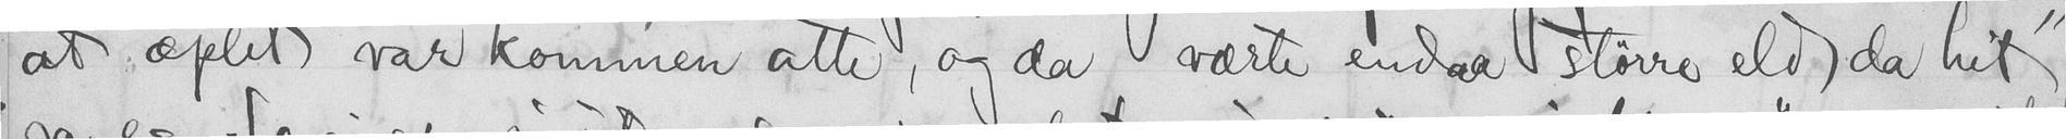at æplet var kommen atte, og da værte endaa større eld da hit",
